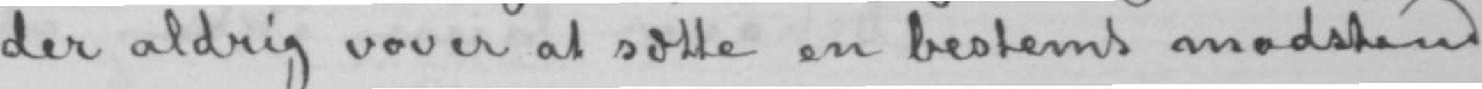der aldrig vover at sætte en bestemt modstand
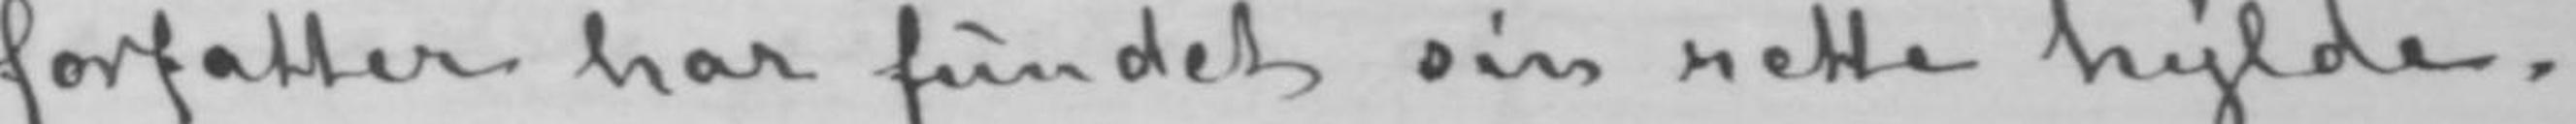forfatter har fundet sin rette hylde.
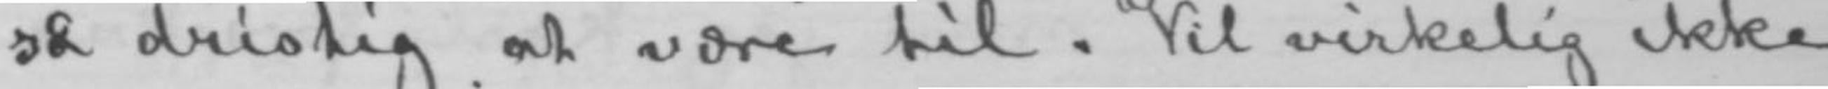så dristig at være til. Vil virkelig ikke
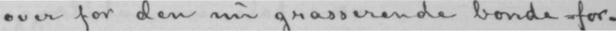over for den nu grasserende bonde-for-
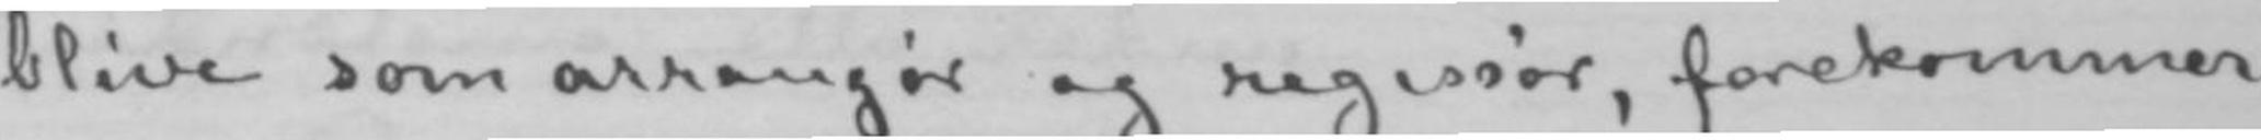blive som arrangør og regissør, forekommer
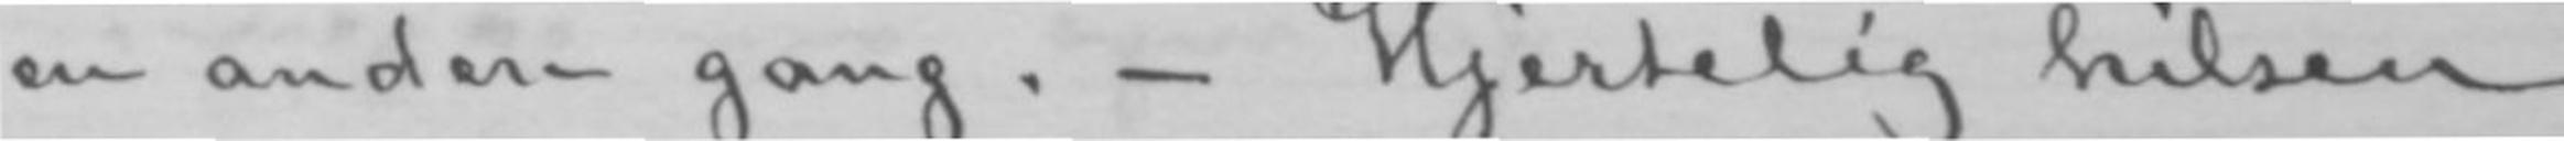en anden gang.- Hjertelig hilsen
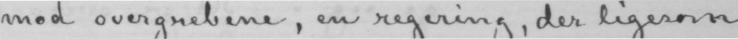mod overgrebene, en regering, der ligesom
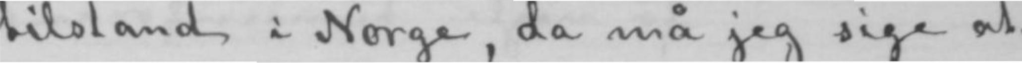tilstand i Norge, da må jeg sige at
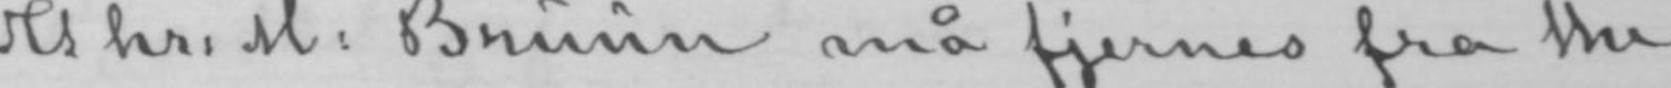At hr: M: Bruun må fjernes fra the-
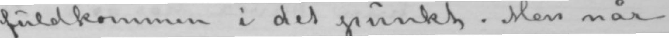fuldkommen i det punkt. Men når
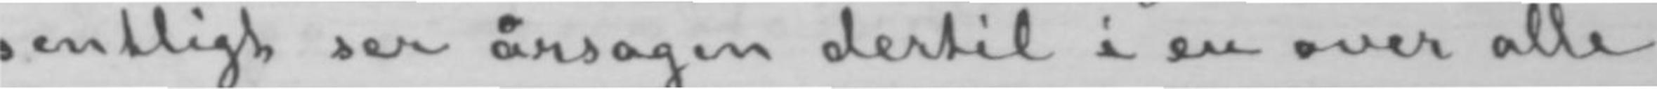sentligt ser årsagen dertil i en over alle
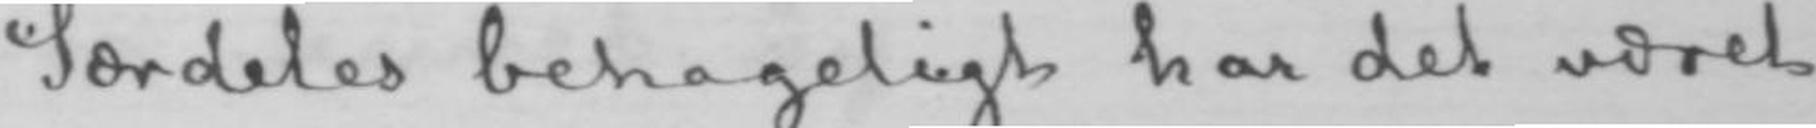Særdeles behageligt har det været
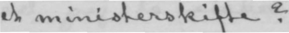et ministerskifte?
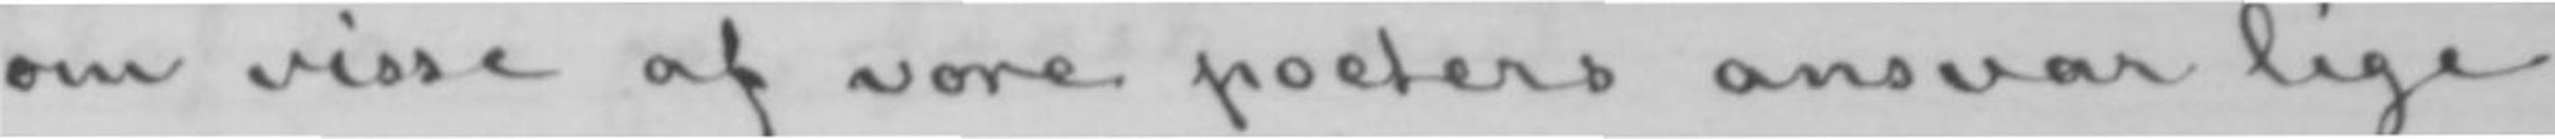om visse af vore poeters ansvar lige
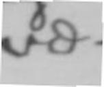væ-
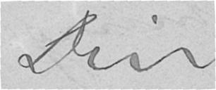Din
